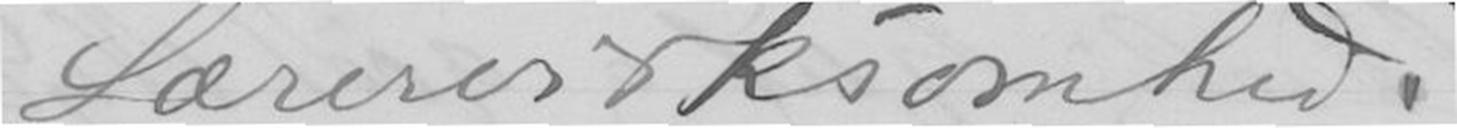Lærervirksomhed.
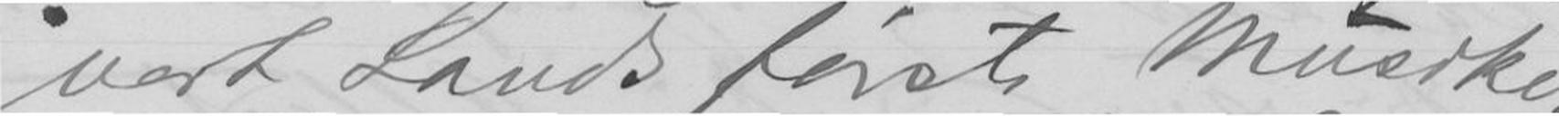vort Lands første Musiker.
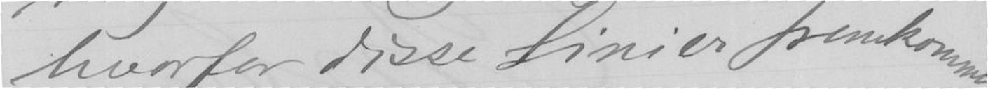hvorfor disse Linier fremkommer.
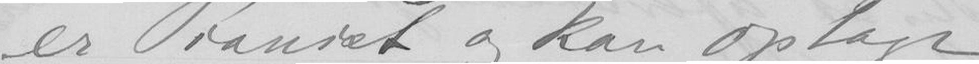er Pianist og kan optage
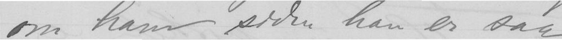om ham siden han er saa
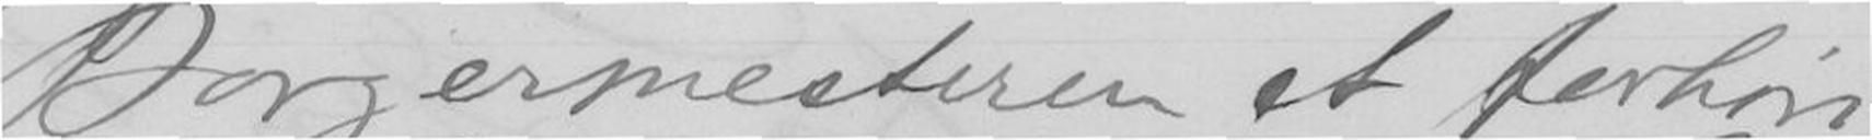Borgermesteren at forhøre
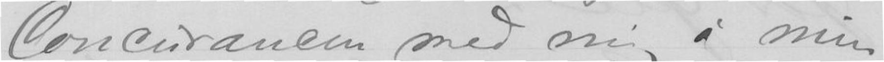Concurancen med mig i min
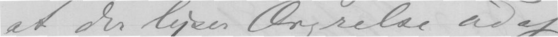at det lyser Ærgrelse ud af
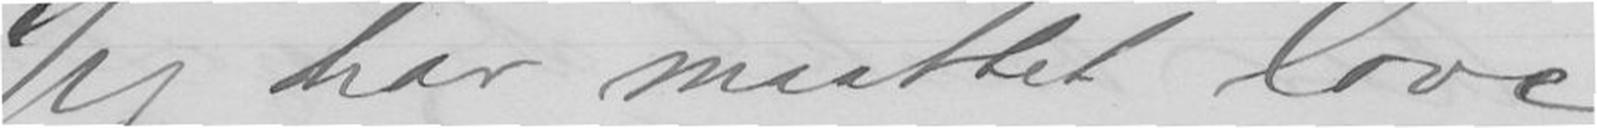Jeg har maattet love
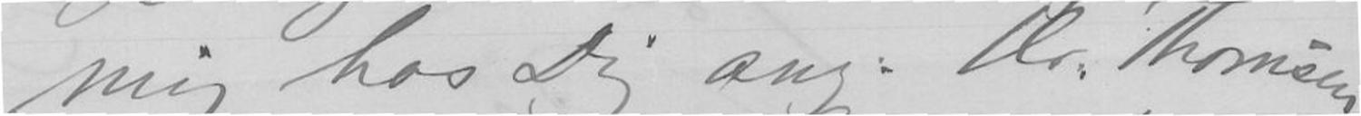mig hos Dig ang. Hr. Thomsen
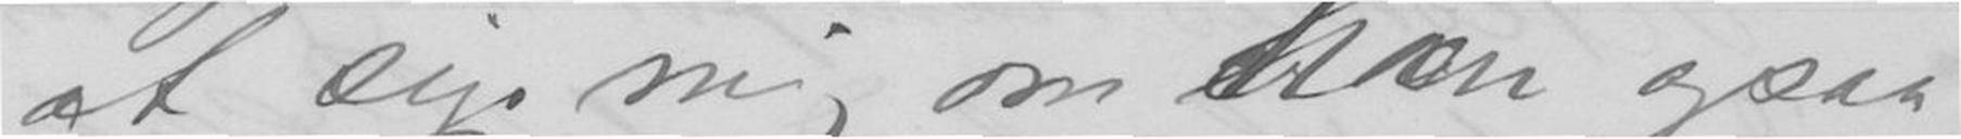at sige mig om han ogsaa
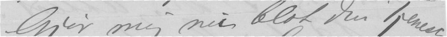Gjør mig nu blot den Tjeneste
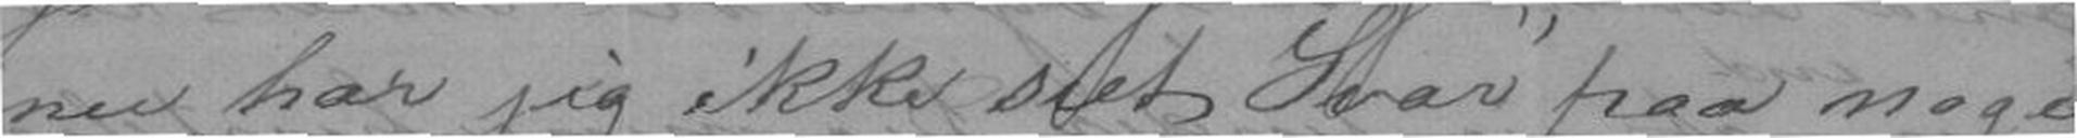nu har jeg ikke seet Svar paa nogen,
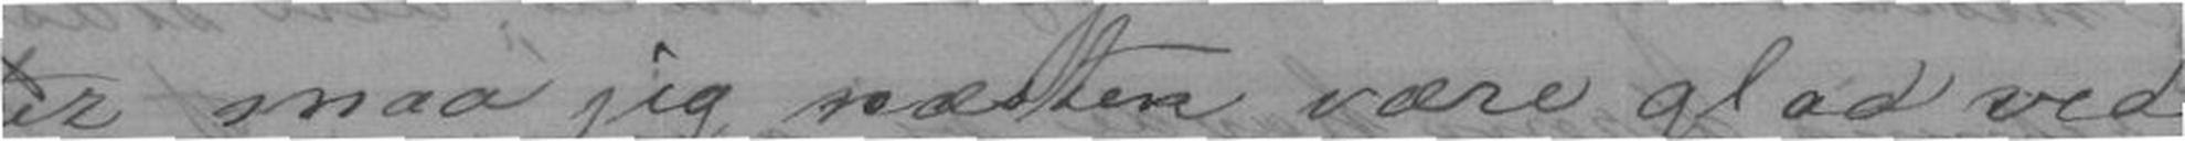ter, maa jeg næsten være glad ved
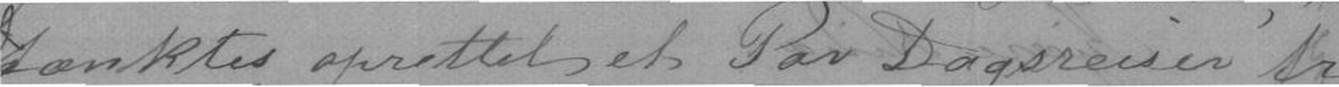tænktes oprettet et Par Dagsreiser fra
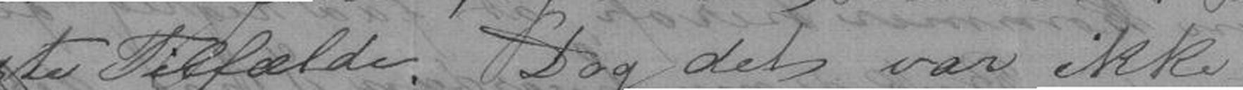ste Tilfælde. Dog det var ikke
